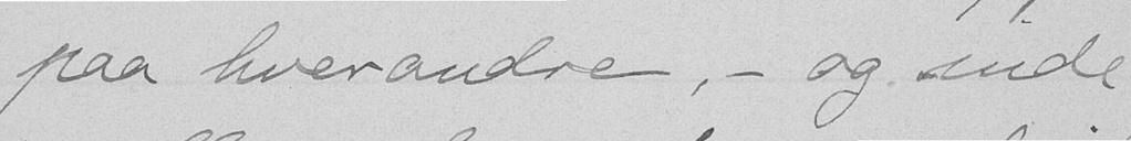paa hverandre, - og inde
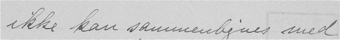ikke kan sammenlignes med
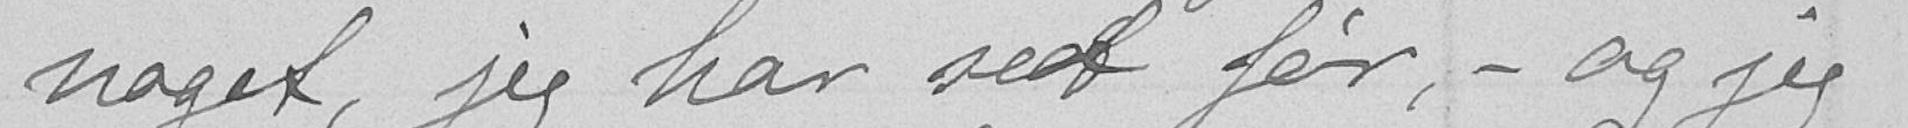noget, jeg har set før,- og jeg
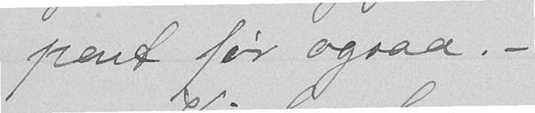pent før ogsaa, -
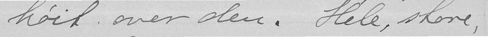høit, over den. Hele, store,
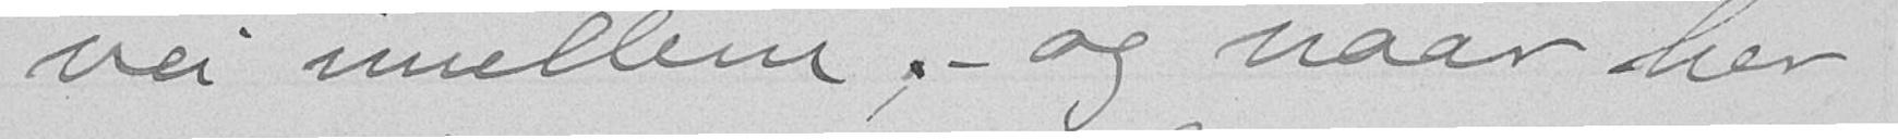vei imellem,- og naar her
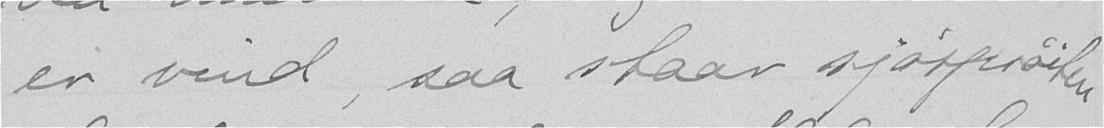er vind, saa staar sjøsprøiten
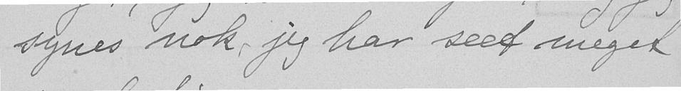synes nok, jeg har seet meget
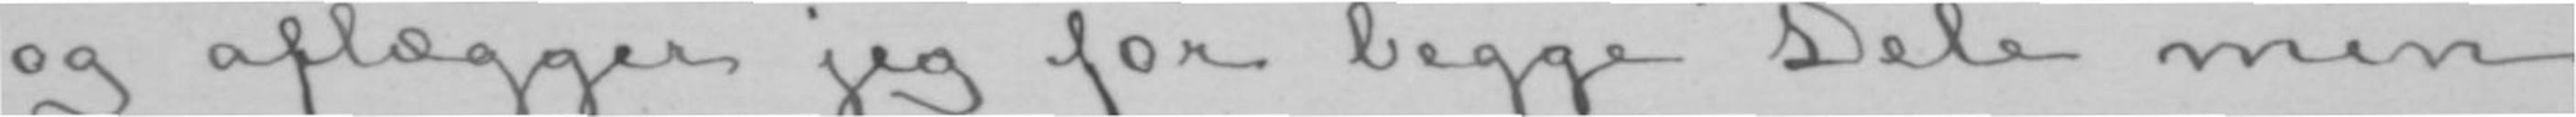og aflægger jeg for begge Dele min
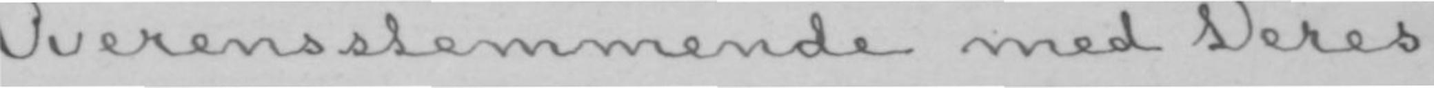Overensstemmende med Deres
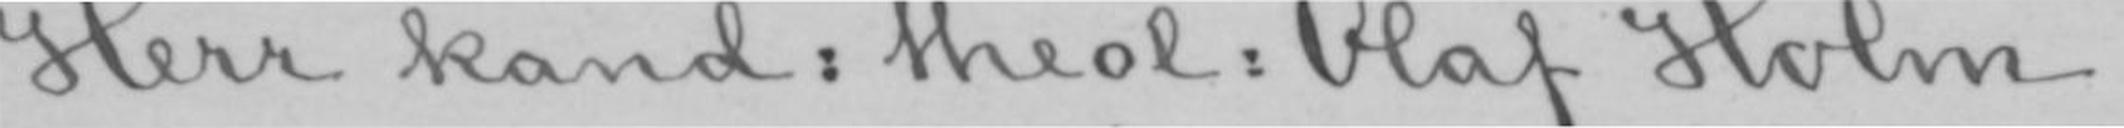Herr kand: theol: Olaf Holm.
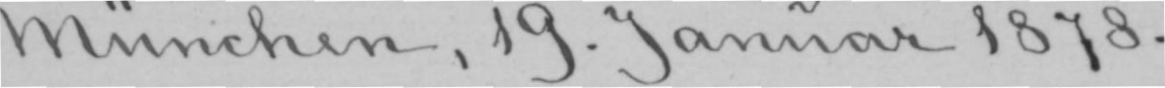München, 19. Januar 1878.
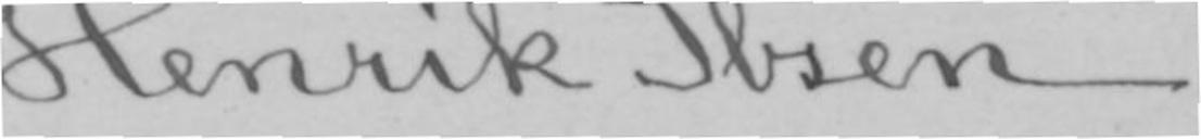Henrik Ibsen.
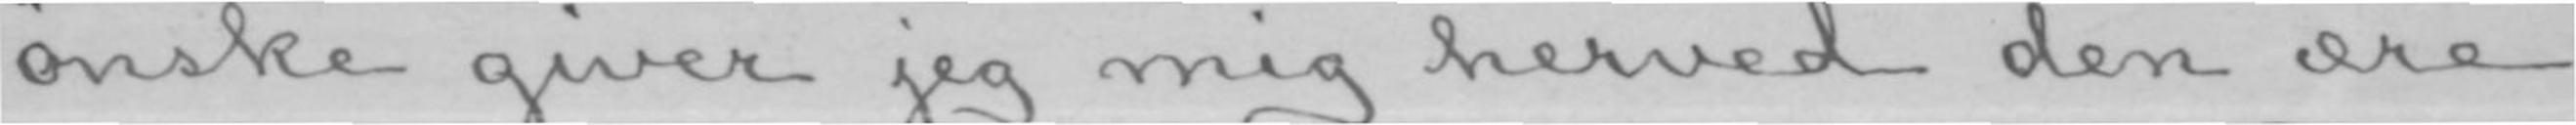ønske giver jeg mig herved den ære
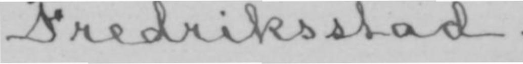Fredriksstad.
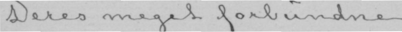Deres meget forbundne
